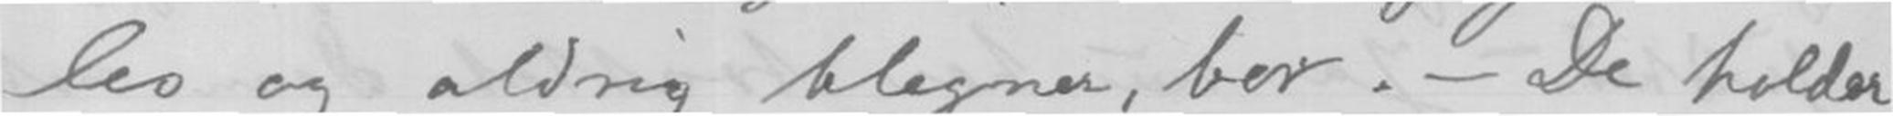les og aldrig blegner, bor. - De holder
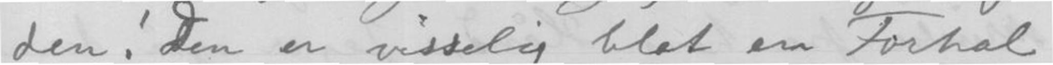den! Den er visselig blot en Forhold
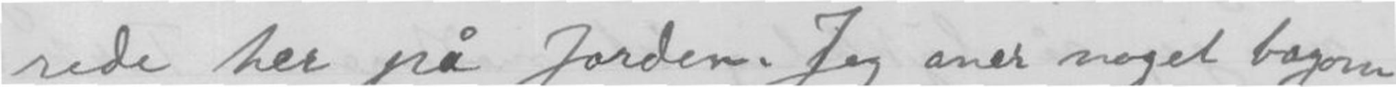rede her på Jorden. Jeg aner noget bagom
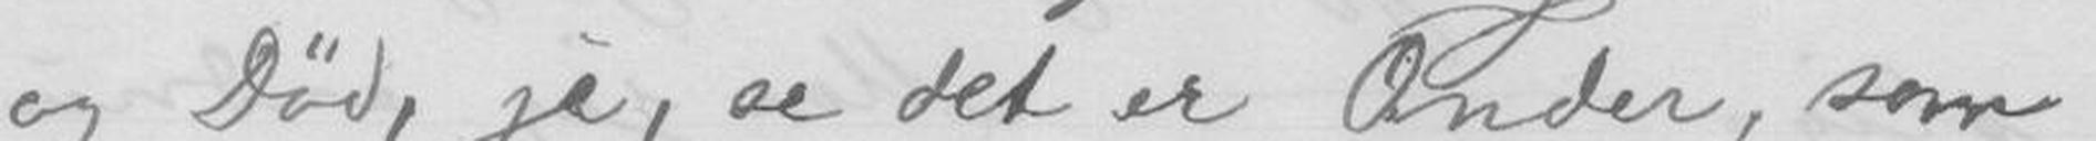og Død, ja, se det er Onder, som det støvet.
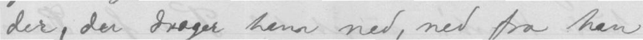der, der drager ham ned, ned fra han
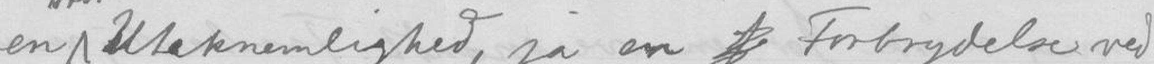en stor Utaknemlighed, ja en f Forbrytelse ved
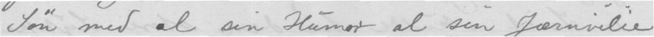Søn med al sin Humor al sin Jernvilie
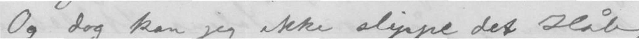Og dog kan jeg ikke slippe det Håb,
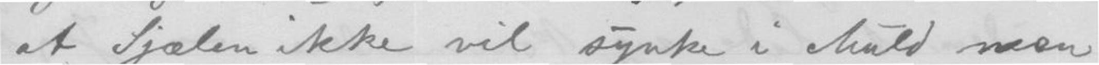at Sjælen ikke vil synke i Muld men
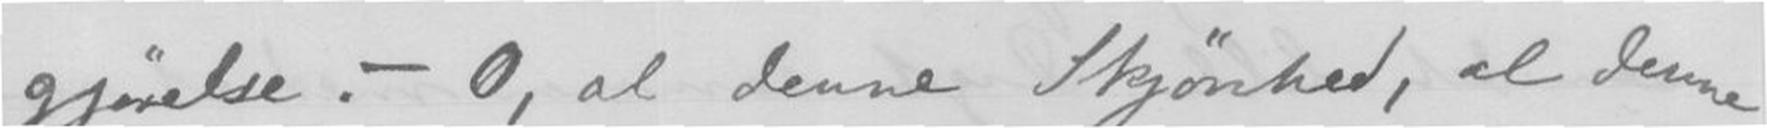gjørelse. - O, al denne Skjønhed, al denne
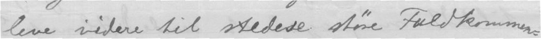leve videre til stedse støre Fuldkommen-
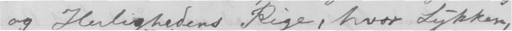og Herlighedens Rige, hvor Lykken,
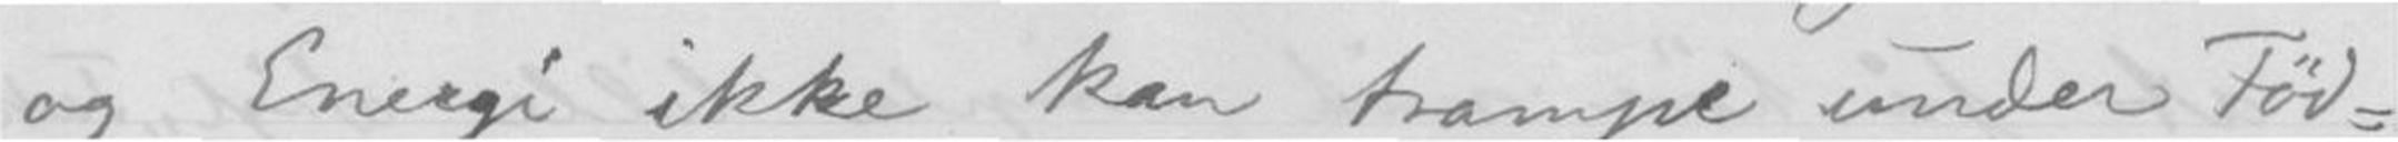og Energi ikke kan trampe under Fød-
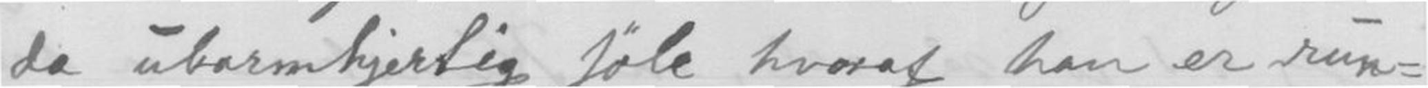da ubarmhjertelig føle hvoraf han er run-
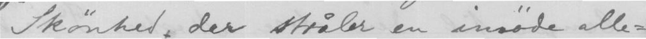Skjønhed, der stråler en imøde alle-
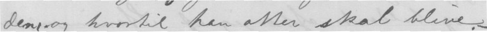den, og hvortil han atter skal blive.
